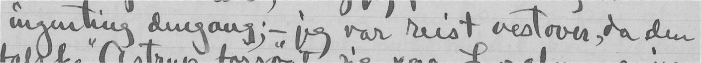ingenting dengang; - jeg var reist vestover, da den
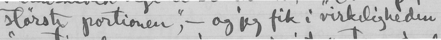største portionen", - og jeg fik i virkeligheden
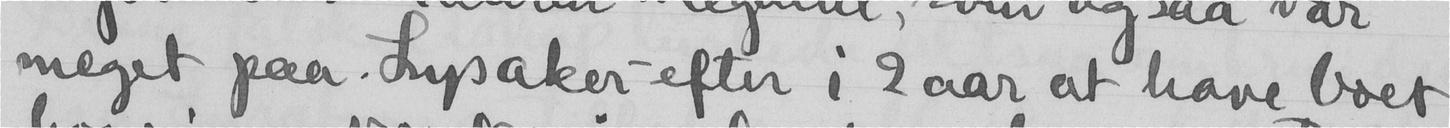meget paa Lyssaker efter i 2 aar at have boet
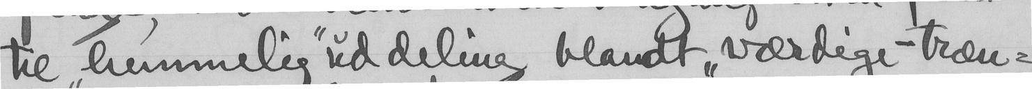til "hemmelig" uddeling blandt "værdige- træn-
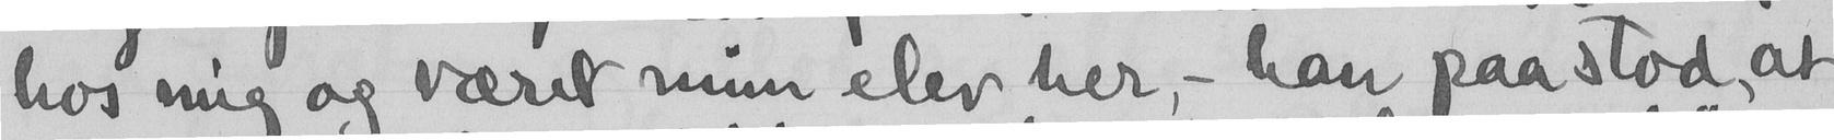hos mig og været min elev her - han paastod, at
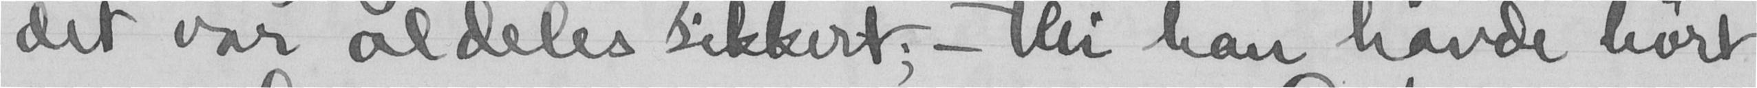det var aldeles sikkert, - thi han havde hørt
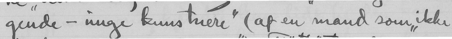gende - unge kunstnere" (af en mand som ikke
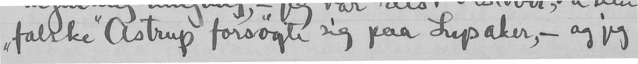"falske" Astrup forsøgte sig paa Lysaker, - og jeg
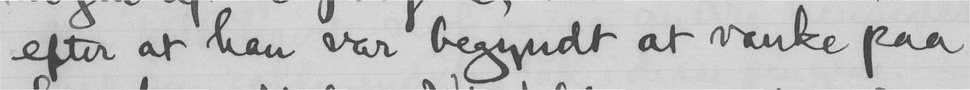efter at han var begyndt at vanke paa
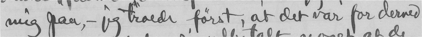mig paa, - jeg troede "først, at det var for derved
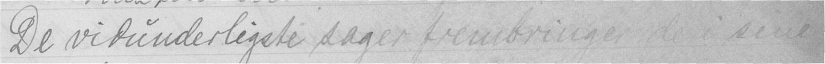De vidunderligste sager frembringer de i sine
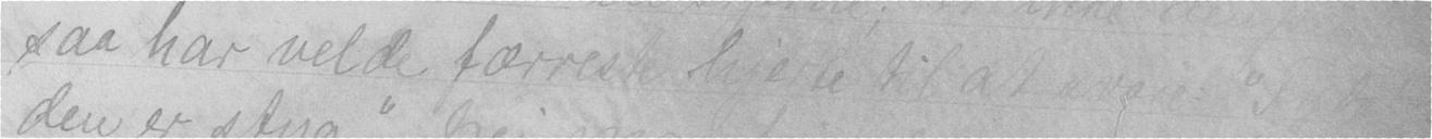saa har vel de færreste hjerte til at svare: "fy da!
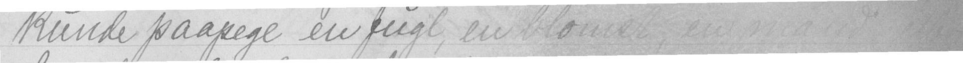kunde paapege en fugl, en blomst, en mand eller
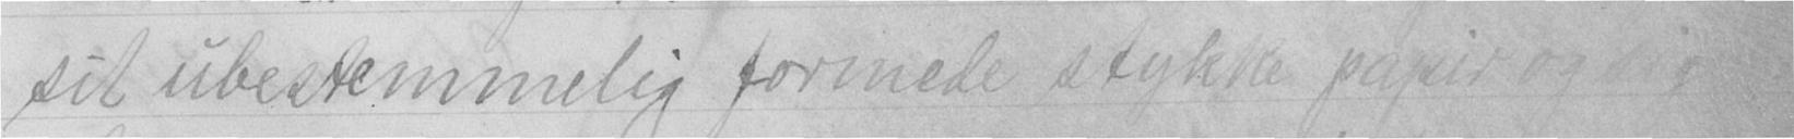sit ubestemmelig formede stykke papir og siger:
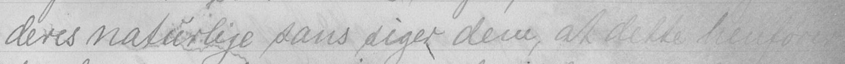deres naturlige sans siger dem, at dette henfører
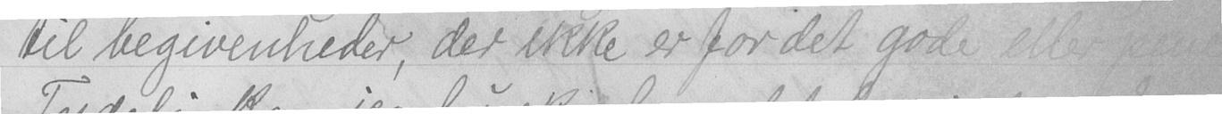til begivenheder, der ikke er for det gode eller pene.
